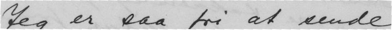Jeg er saa fri at sende
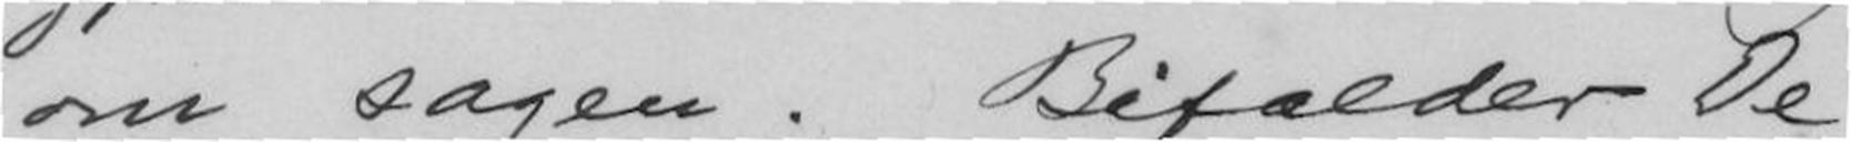om sagen. Bifalder De
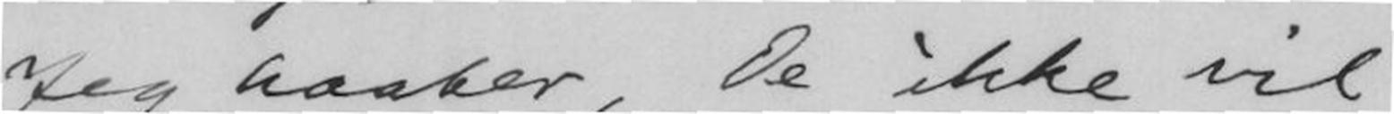Jeg haaber, De ikke vil
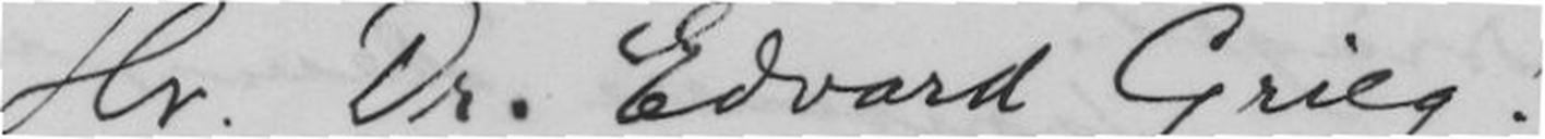Hr. Dr. Edvard Grieg !
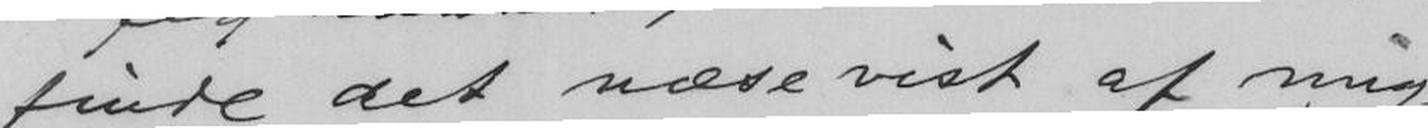finde det næsevist af mig,
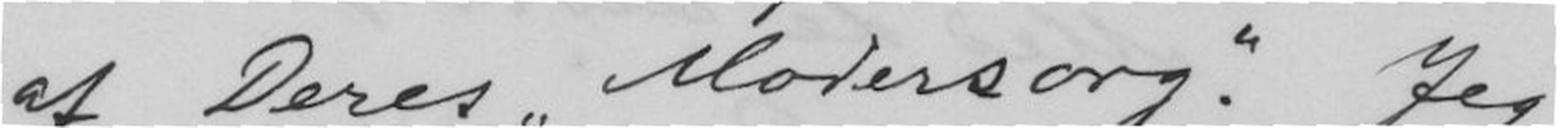af Deres "Modersorg". Jeg
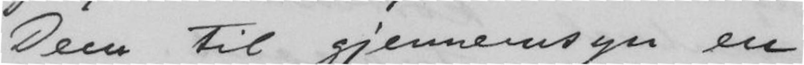Dem til gjennemsyn en
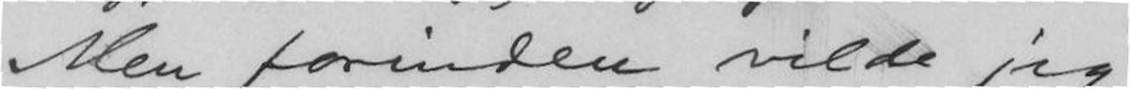Men forinden vilde jeg
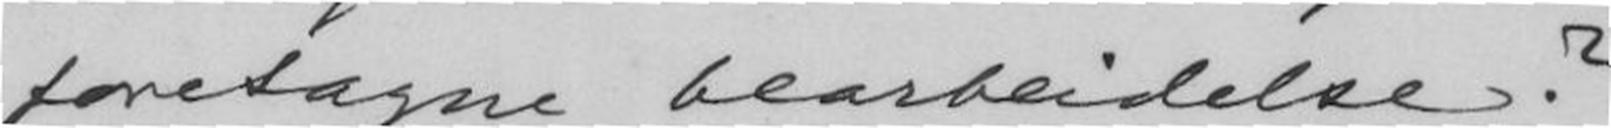foretagne bearbeidelse?
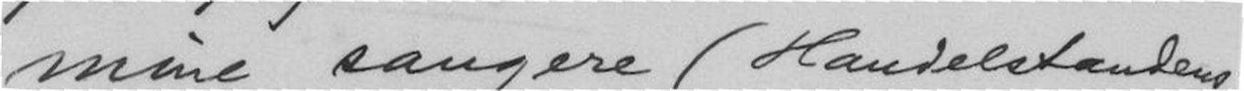mine sangere (Handelstandens
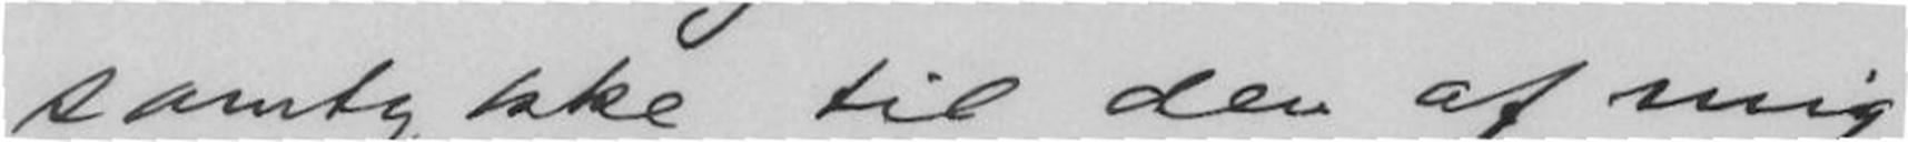samtykke til den af mig
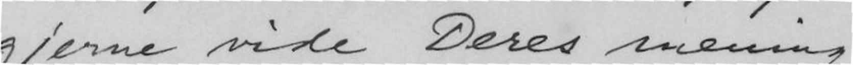gjerne vide Deres mening
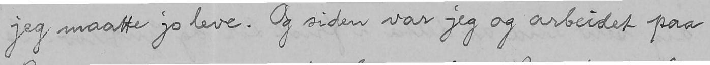jeg maatte jo leve. Og siden var jeg og arbeidet paa
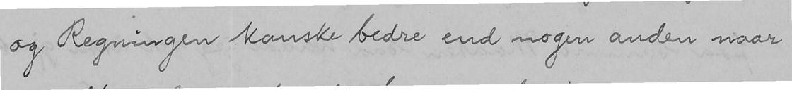og Regningen kanske bedre end nogen anden naar
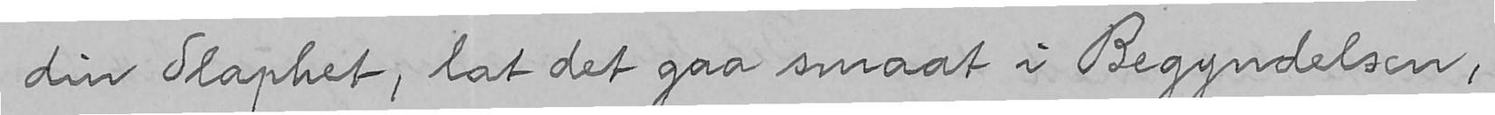din Slaphet, lat det gaa smaat i Begyndelsen,
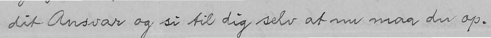dit Ansvar og si til dig selv at nu maa du op.
